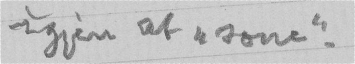igjen at "sone".
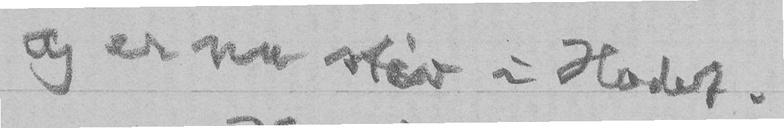og er nu stiv i Hodet.
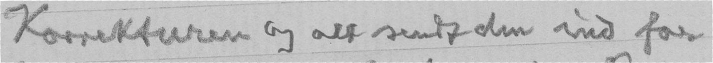Korrekturen og alt sendt den ind for
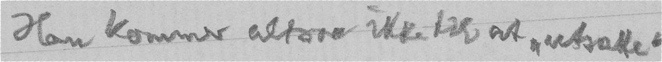Han kommer altsaa ikke til at "utsætte"
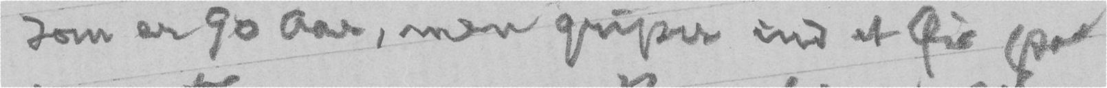som er 90 Aar, men griper ind et Øie paa
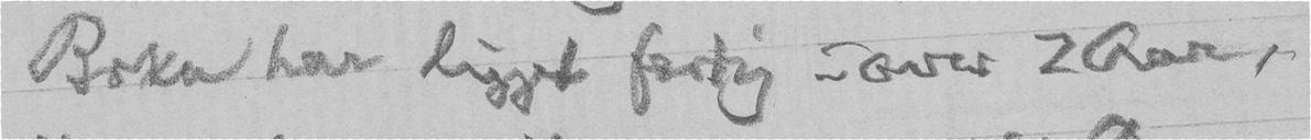Boka har ligget færdig i over 2 Aar,
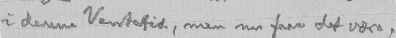i denne Ventetid, men nu faar det være,
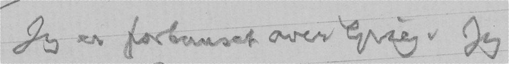Jeg er forbauset over Grieg. Jeg
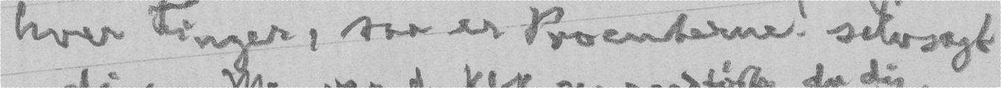hver Finger, saa er Procenterne selvsagt
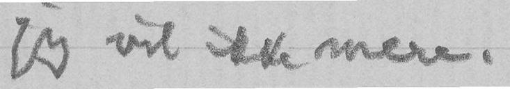jeg vil ikke mere.
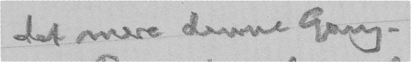det mere denne Gang.
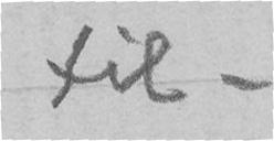til.
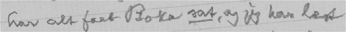har alt faat Boka sat, og jeg har læst
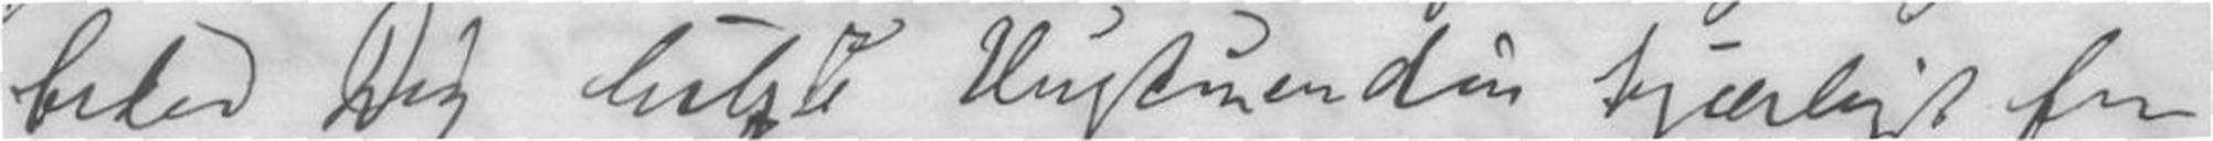beder Dig hilse Hustruen din hjærteligst fra
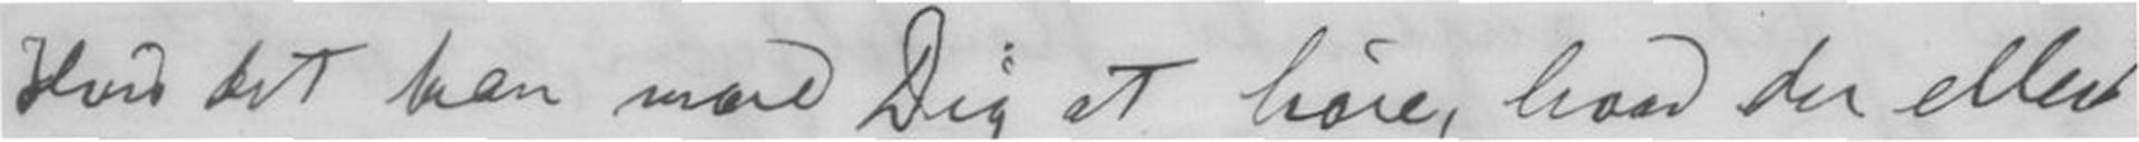Hvis det kan more Dig at høre, hvad det ellers
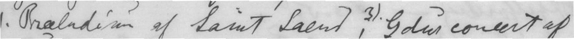1. Præludium af Saint Saens, 3). Gdur concert af
